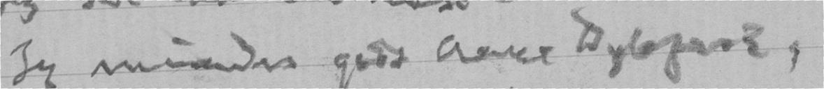Jeg mindes godt Arne Dybfest,
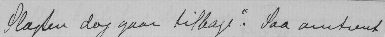Slægten dog gaar tilbage". Saa omtrent
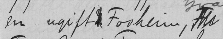en ugift  Fosheim,
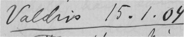Valdris 15.1.04
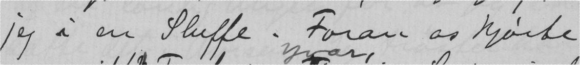jeg i en Sluffe. Foran os kjørte
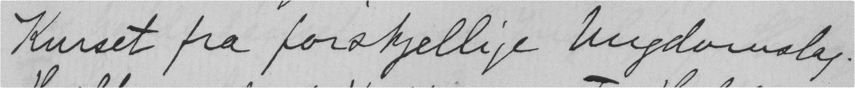Kurset fra forskjellige Ungdomslag.
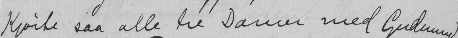Kjørte saa alle tre Damer med Gudmund
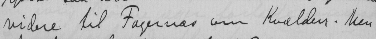videre til Fagernæs om Kvælden. Men
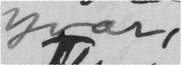Ivar,
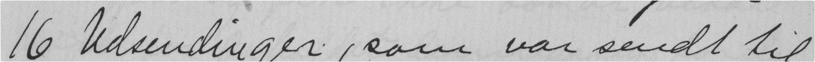16 Udsendinger, som var sendt til
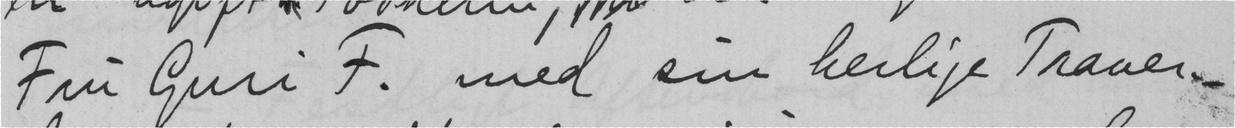Fru Guri F. med sin herlige Traver-
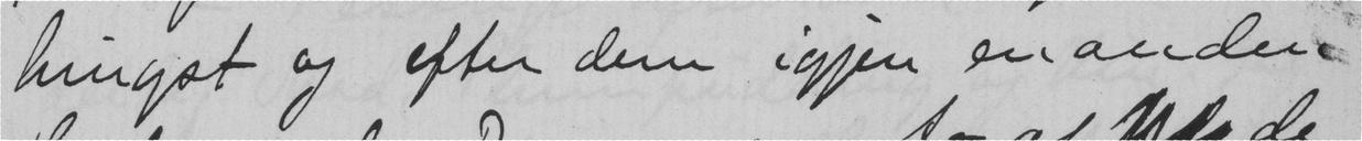hingst og efter dem igjen en anden
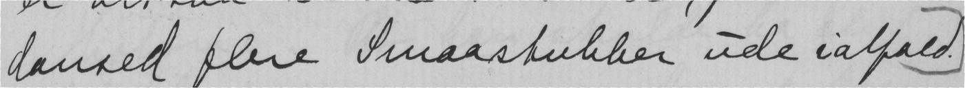dansed flere Smaastubber ude ialfald.
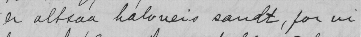er altsaa halvveis sandt, for vi
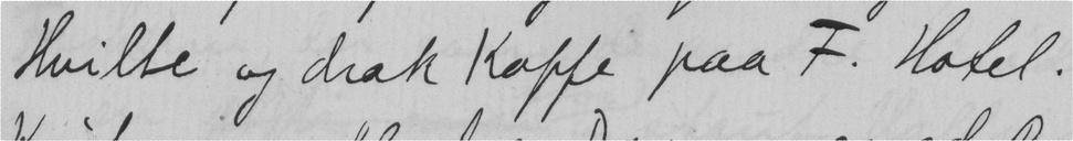Hvilte og drak Kaffe paa F. Hotel.
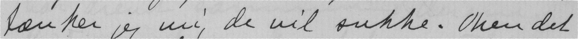tænker jeg mig de vil sukke. Men det
